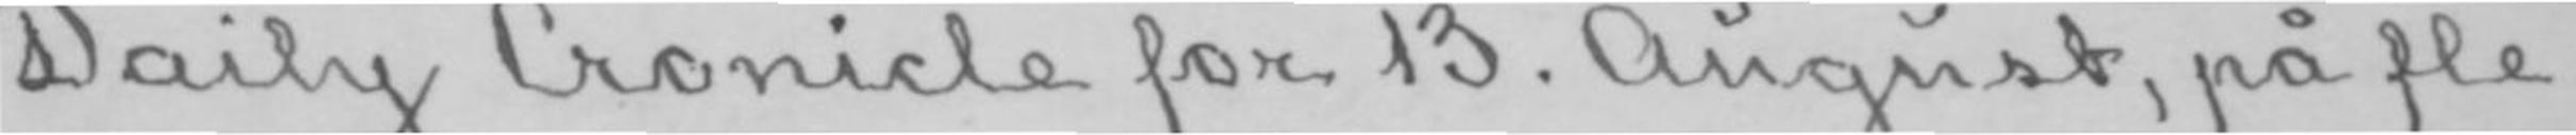Daily Cronicle for 13. August, på fle-
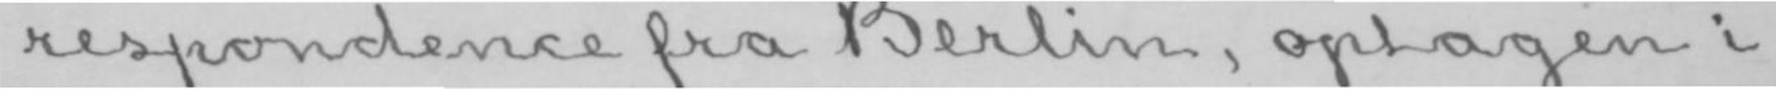respondence fra Berlin, optagen i
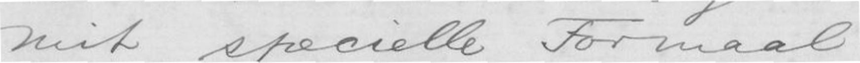mit spesielle Formaal,
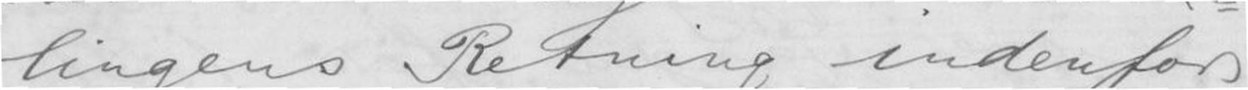linges Retning indenfor
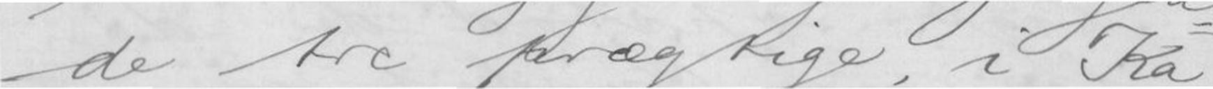de tre prægtige, i Ka
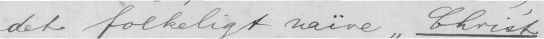det folkeligt naïve Christ
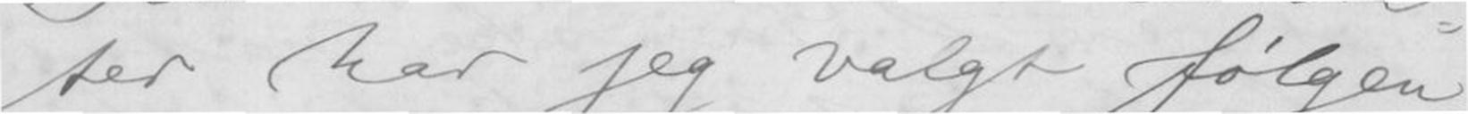ter har jeg valgt følgen
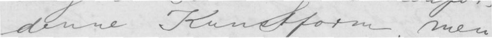denne Kunstform, men
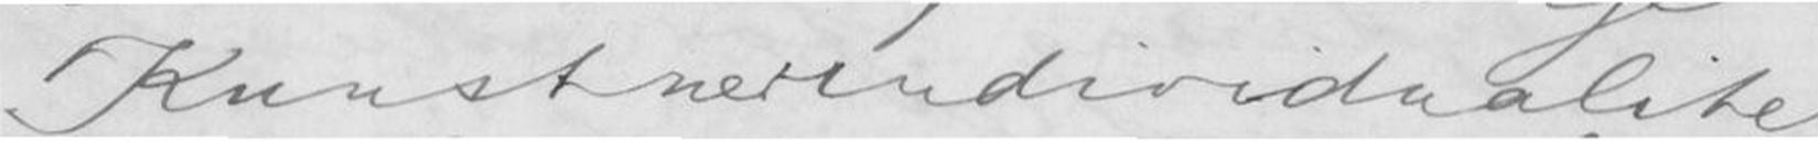Kunstnerindividualite
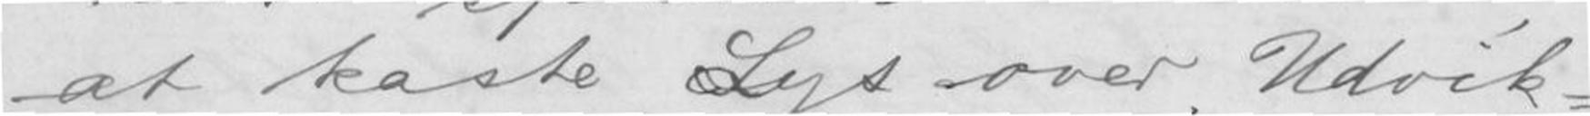at kaste Lys over Udvik-
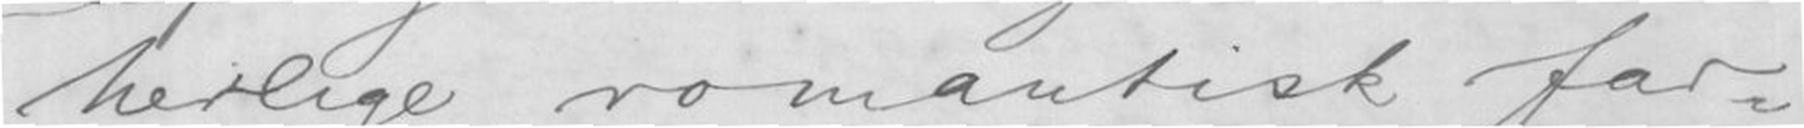herlige, romantisk far-
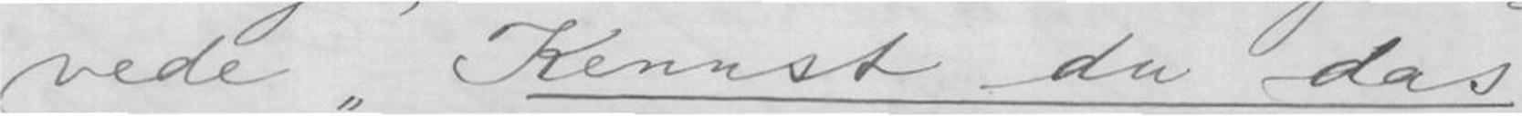vede Kennst du das
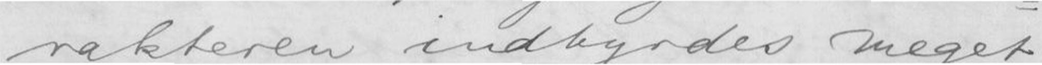rakteren indkyndes meget
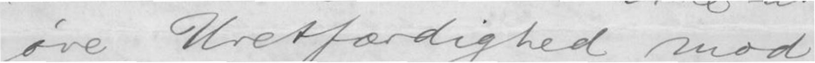øve Uretfærdighed mod
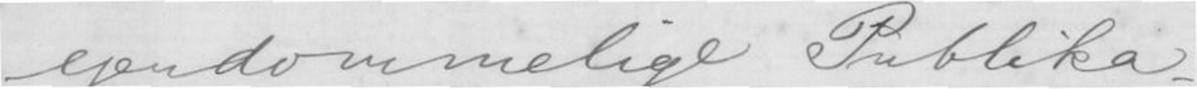ejendommelige Publika-
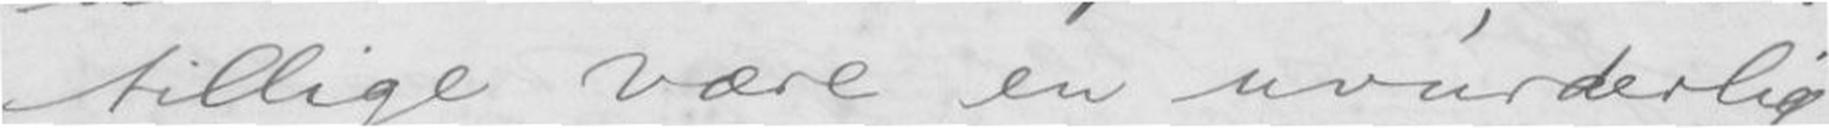tillige være en uvurderlig
